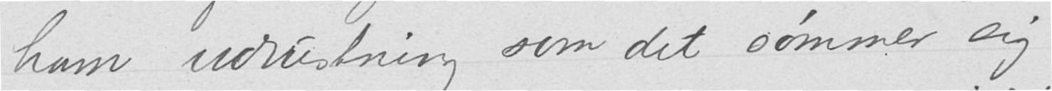ham udrustning som det sømmer sig
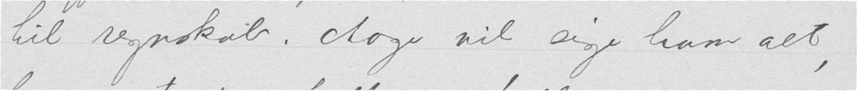til regnskab. Aage vil sige ham alt,
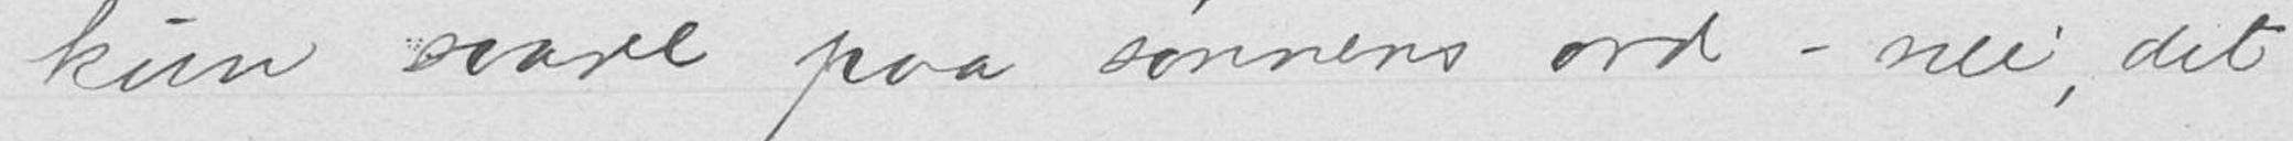kun svare paa sønnens ord – nei, det
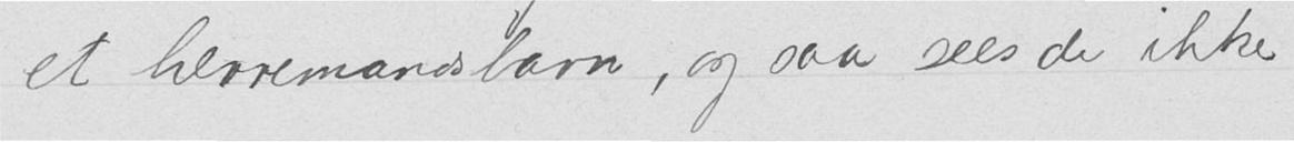et herremandsbarn, og saa sees de ikke
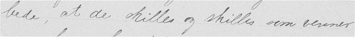bede, at de skilles og skilles som venner
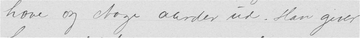hove og Aage aander ud. Han giver
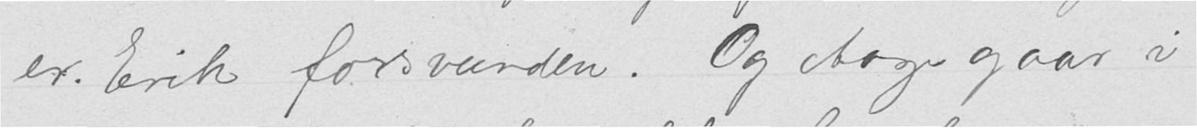er Erik forsvunden. Og Aage gaar i
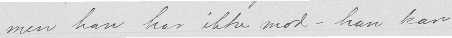men han har ikke mod – han kan
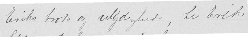Eriks trods og ulydighed, ti Erik
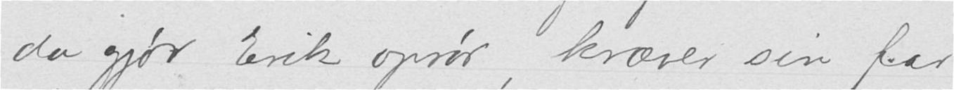da gjør Erik oprør, kræver sin far
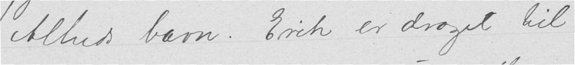Alheds barn. Erik er draget til
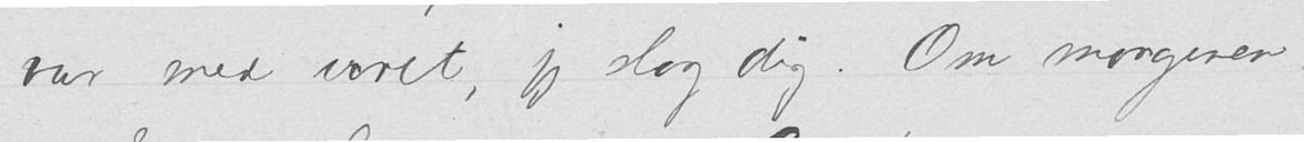var med uret, jeg slog dig. Om morgenen
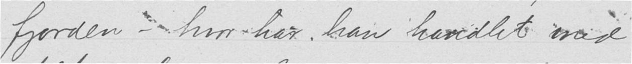fjorden – hvor har han handlet mod
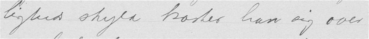ligheds skyld kaster han sig over
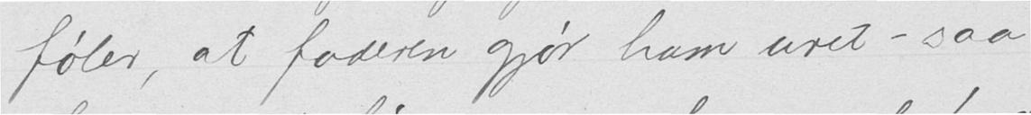føler, at faderen gjør ham uret – saa
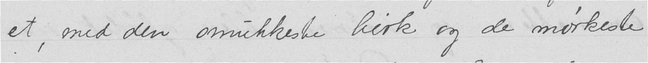et, med den smukkeste birk og de mørkeste
